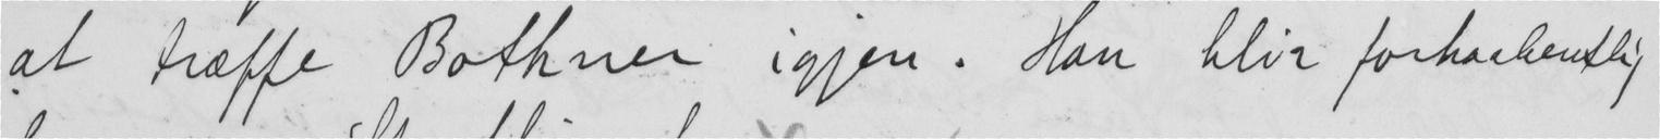at træffe Bothner igjen. Han blir forhaabentlig
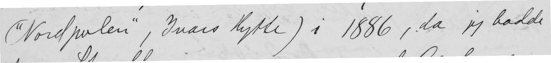("Nordpolen", Ivars Hytte) i 1886, da jeg bodde
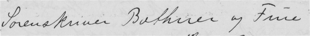Sorenskriver Bothner og Frue
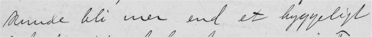kunde bli mer end et hyggeligt
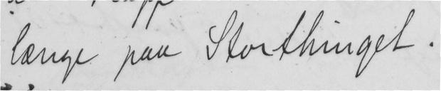længe paa Storthinget.
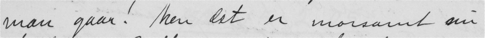man gaar! Men det er morsomt nu
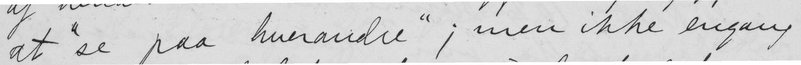at "se paa hverandre"; men ikke engang
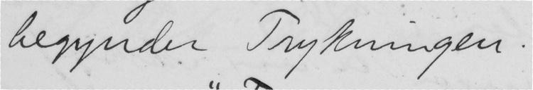begynder Trykningen.
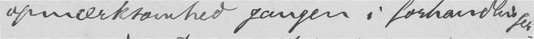opmærksomhed gangen i forhandlinger-
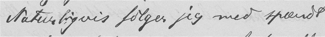Naturligvis følger jeg med spendt
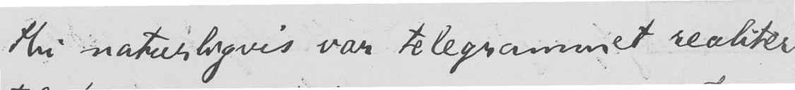thi naturligvis var telegrammet realiter
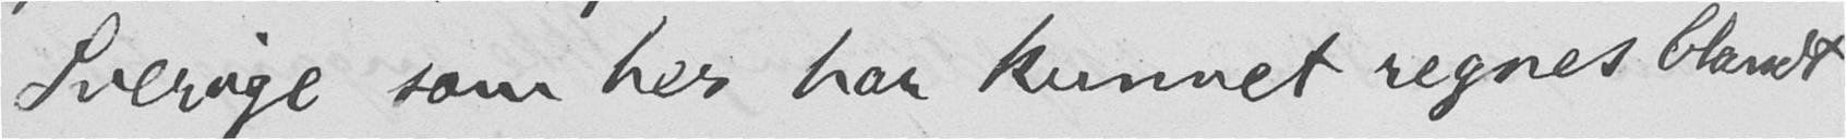Sverige som her har kunnet regnes blandt
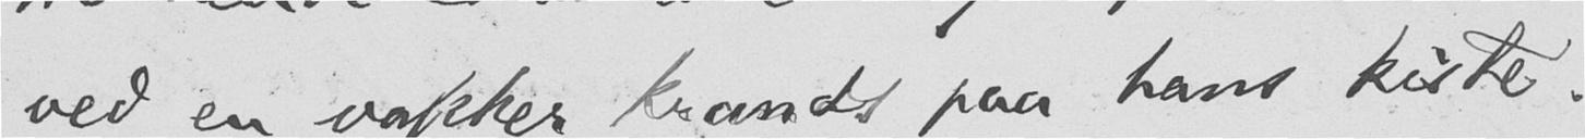ved en vakker krands paa hans kiste.
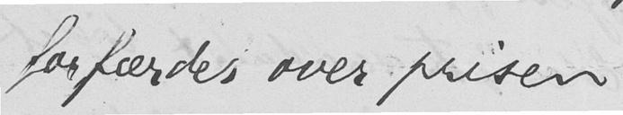forfærdes over prisen.
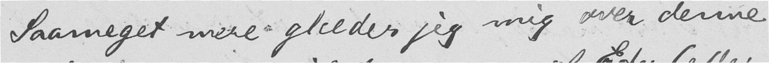Saameget mere glæder jeg mig over denne
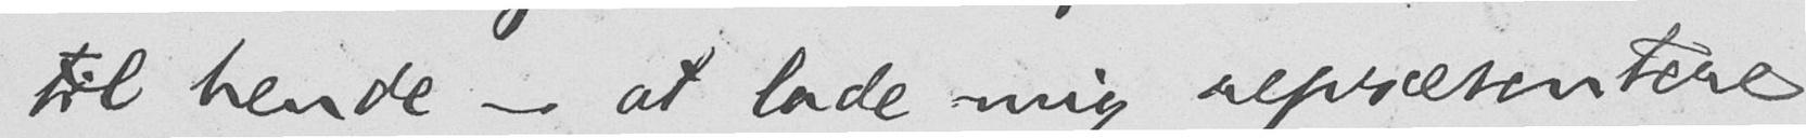til hende - at lade mig repræsentere
